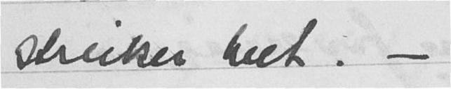streiker helt. -
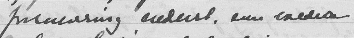forurensing nederst, som holder
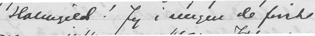Holmgild. Jeg i sengen de første
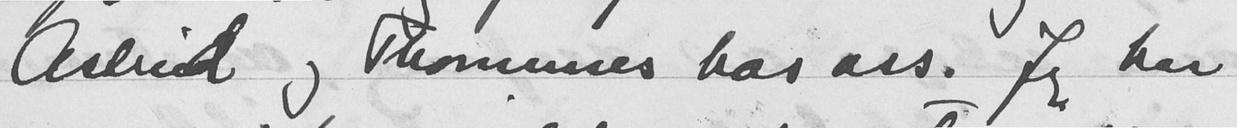Astrid og Thommes hos oss. Jeg har
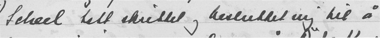Scheel tatt skrittet og besluttet mig til å
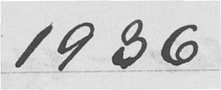1936
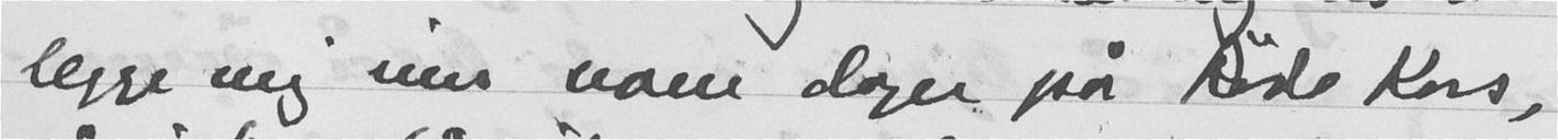legge mig inn noen dager på Røde Kors,
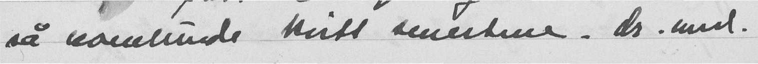så noenlunde kvitt smertene. Dr. med.
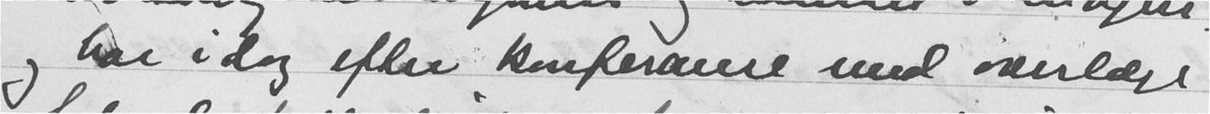og har i dag efter konferanse med overlæge
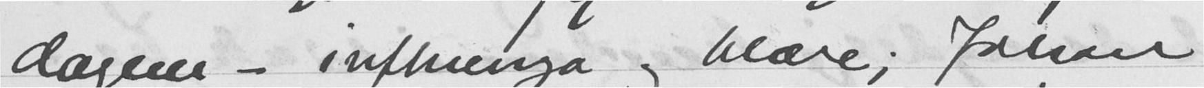dagene - influenza og blære; Johan
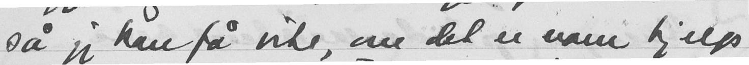så jeg kan få vite, om det er noen hjelp
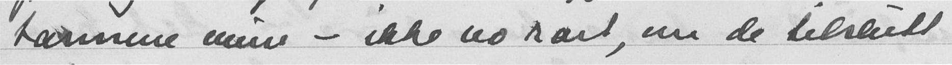tarmene mine - ikke no rart, om de tilslutt
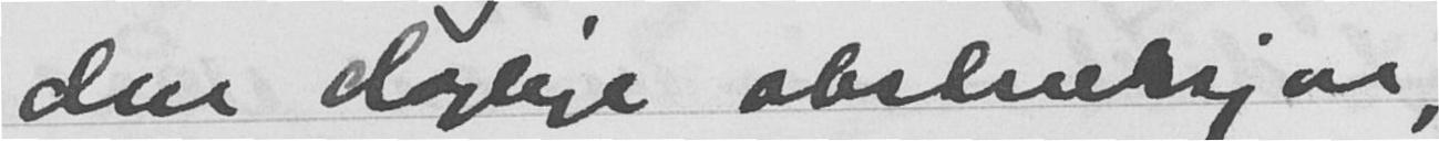den daglige obstieksjas,
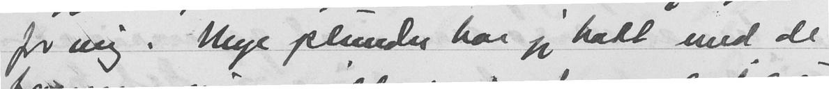for mig. Mye plunder har jeg hatt med de
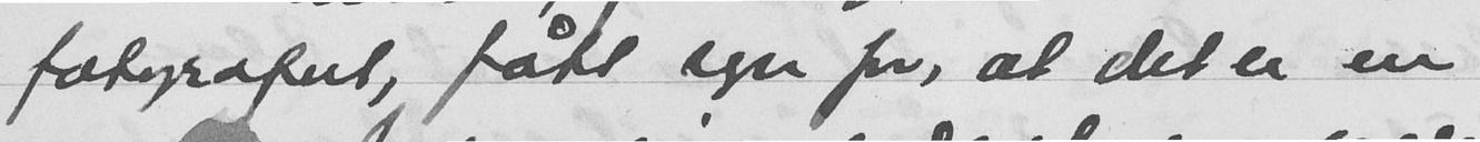fotografert, fått egen for, at det er en
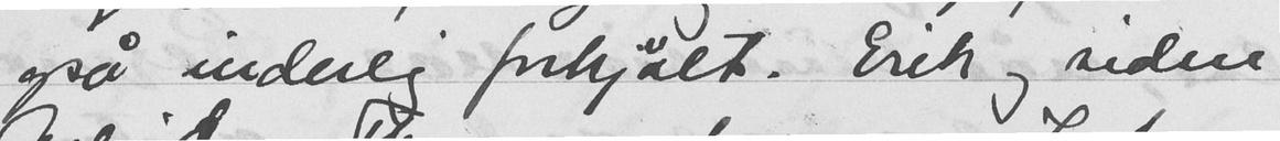også inderlig forkjølt. Erik og siden
